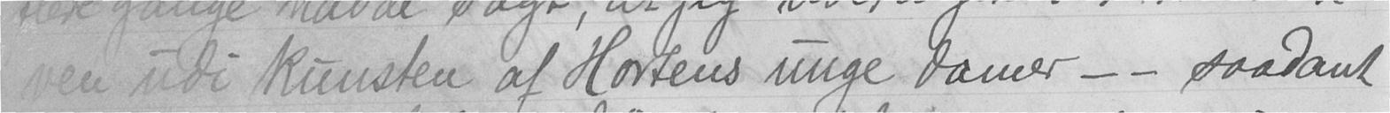ven udi kunsten af Hortens unge damer - - saadant
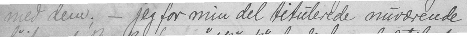med dem; - Jeg for min del titulerede nuværende
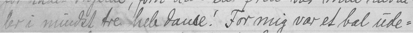ler i mindst tre hele danse! For mig var et bal ude-
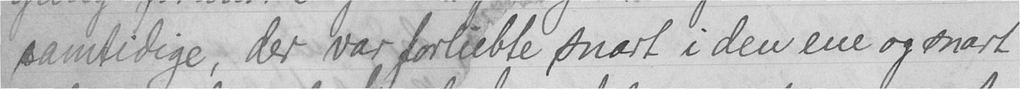samtidige, der var forliebte snart i den ene og snart
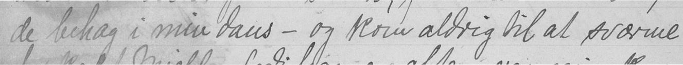de behag i min dans - og kom aldrig til at sværme
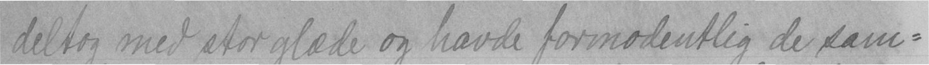deltog med stor glæde og havde formodentlig de sam-
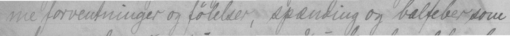me forventninger og følelser, spænding og balfeber som
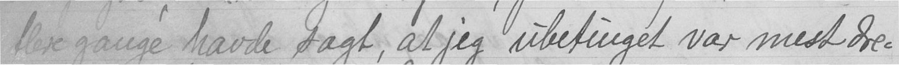flere gange havde sagt, at jeg ubetinget var mest dre-
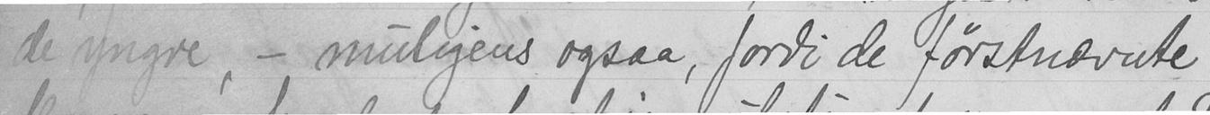de yngre, - muligens ogsaa, fordi de forstnævnte
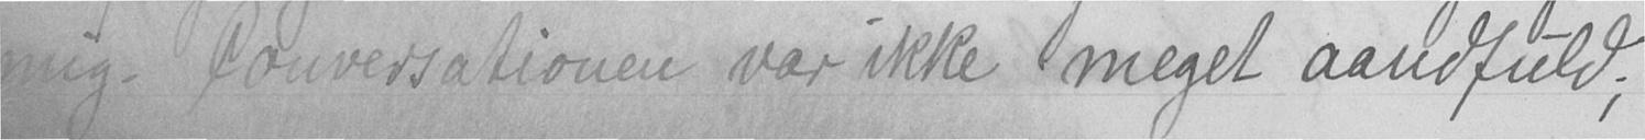mig. Conversationen var ikke meget aandfuld;
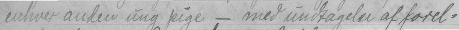enhver anden ung pige - med undtagelse af forel-
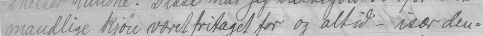mandlige kjøn været fritaget for, og altid - især den-
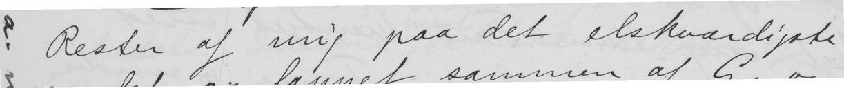Rester af mig paa det elskværdigste
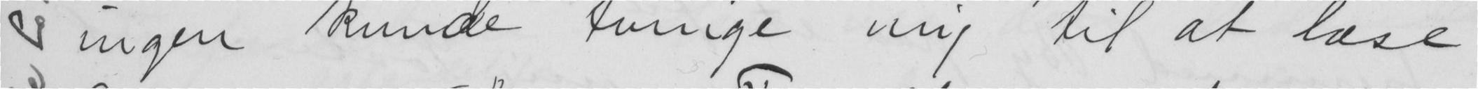ingen kunde tvinge mig til at læse
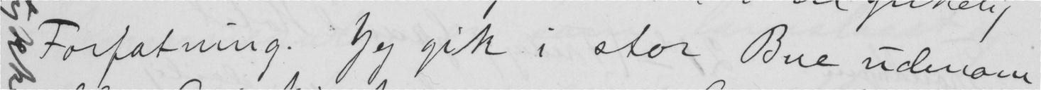Forfatning. Jeg gik i stor Bue udenom
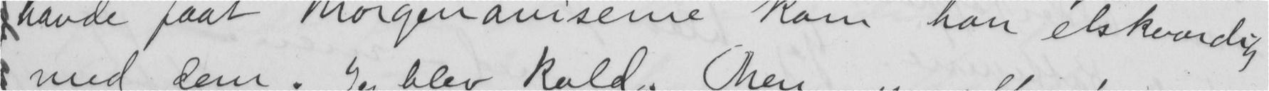havde faat Morgenaviserne kom han elskværdig
